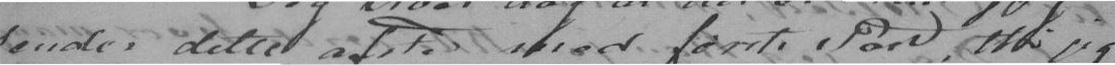sender dette afsted med første Post, thi jeg
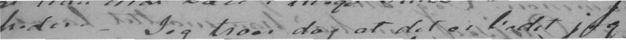heder. - Jeg troer dog at det er bedst jeg
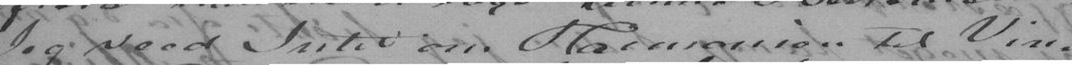Jeg veed Intet om Harmonien til Vin-
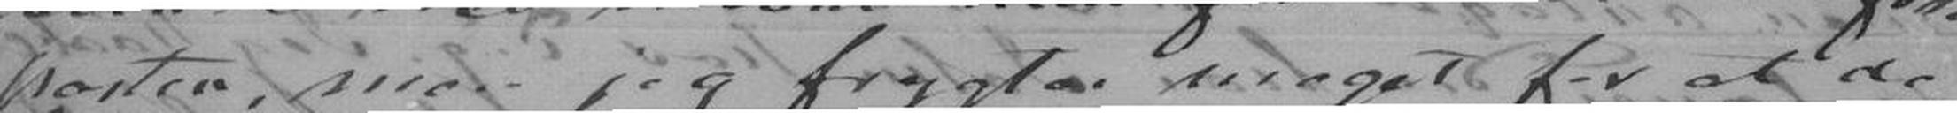posten, men jeg Frygter meget for at de
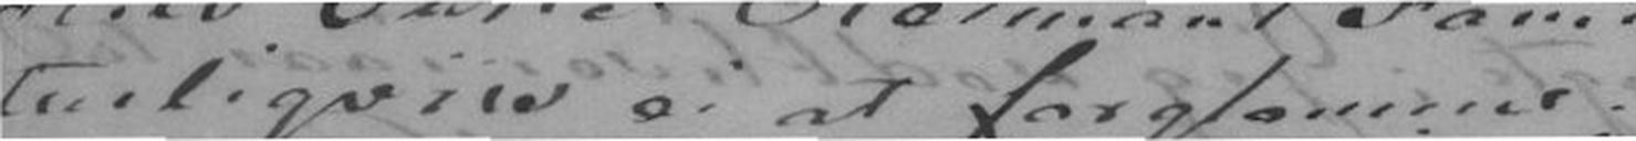turligviis ei for at glemme.
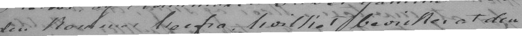den kommer herfra, hvilket bevirker at den
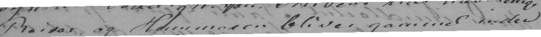Reiser, og Hummeren bliver gammel inden
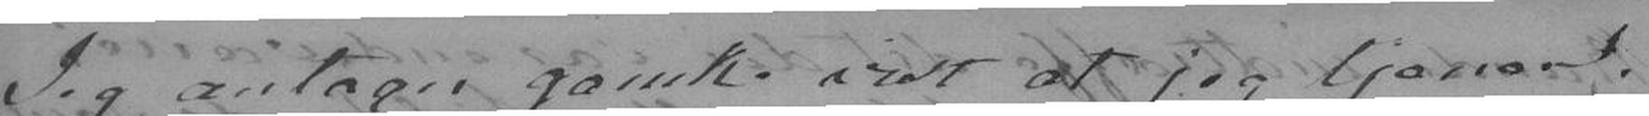Jeg antager ganske vist at jeg tjener
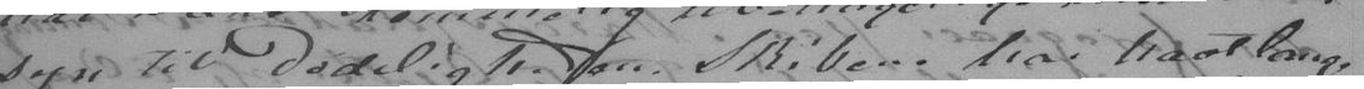syn til Dødeligheden. Skibene har havt lange
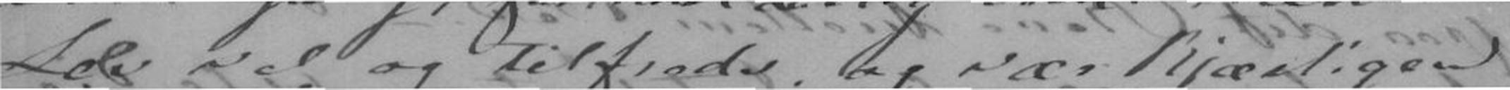Lev vel og tilfreds, og vær kjærligen
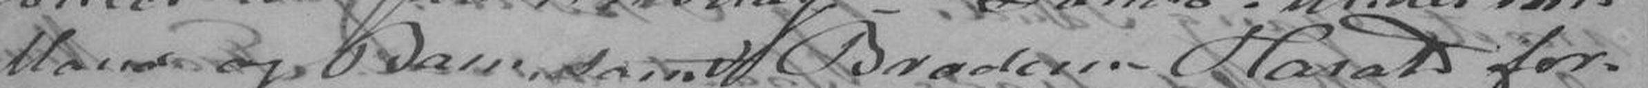Mand og Barn, samt Broderen Harald for-
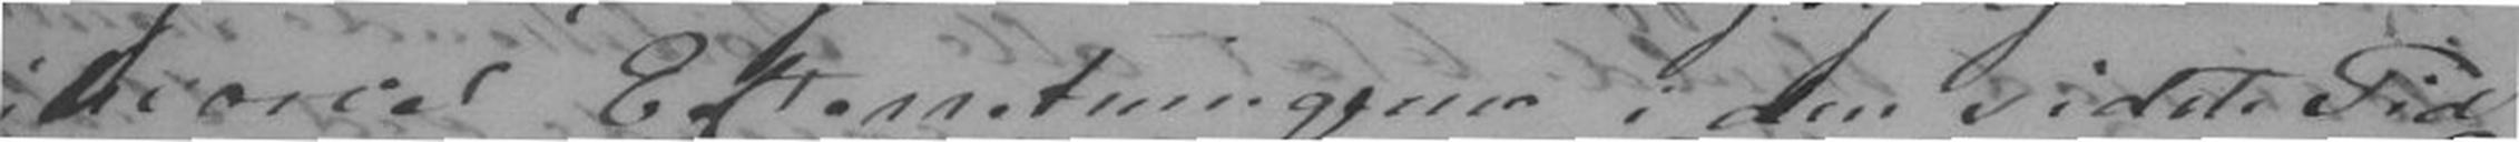ihvorvel Efterretningerne i den sidste Tid
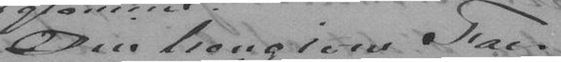Din hengivne Faer
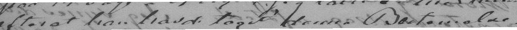efterat han havde taget denne Bestæmmelse.
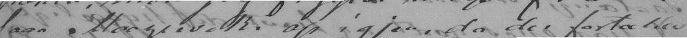faar Maczewski op igjen da der fortælles
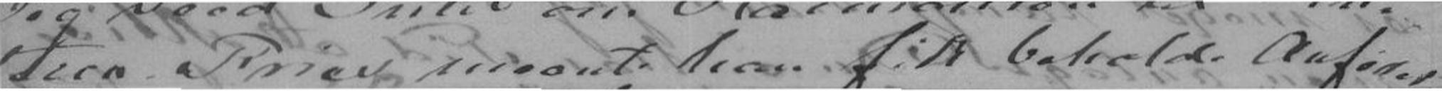teren. Fries meente han fik beholde Anførel
